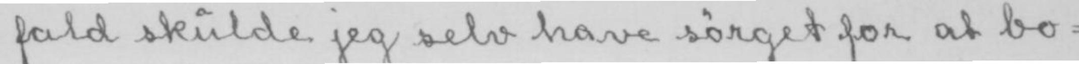fald skulde jeg selv have sørget for at bo-
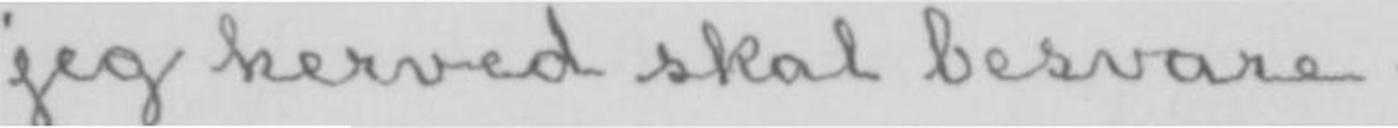jeg herved skal besvare.
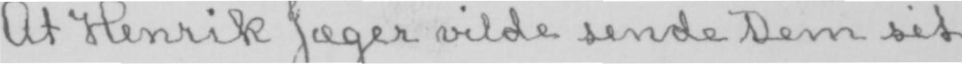At Henrik Jæger vilde sende Dem sit
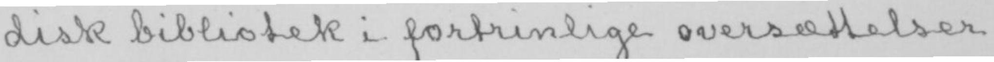disk bibliotek i fortrinlige oversættelser
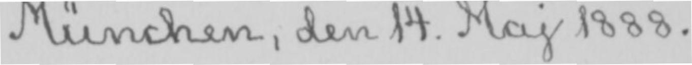München, den 14. Maj 1888.
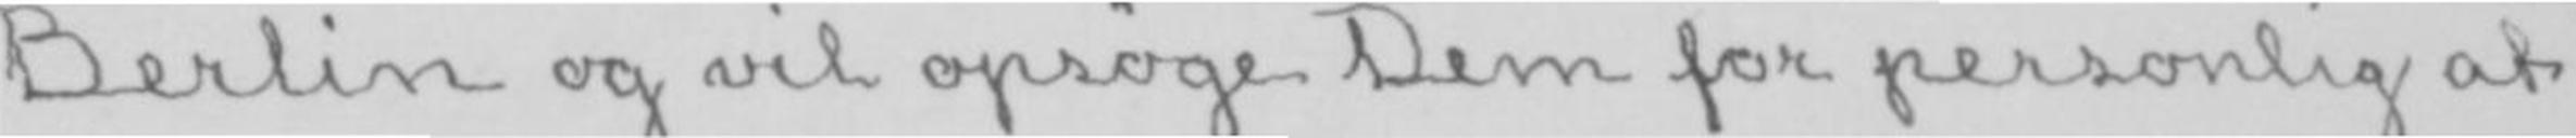Berlin og vil opsøge Dem for personlig at
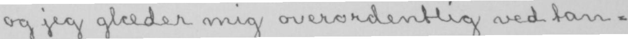og jeg glæder mig overordentlig ved tan-
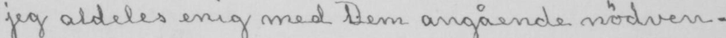jeg aldeles enig med Dem angående nødven-
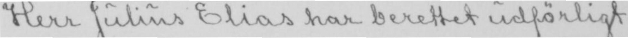Herr Julius Elias har berettet udførligt
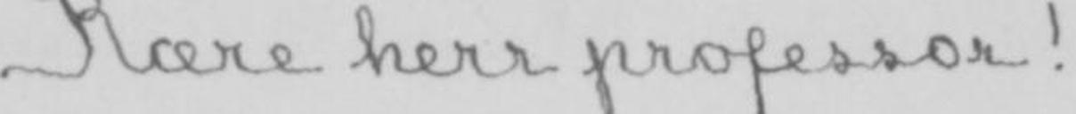Kære herr professor!
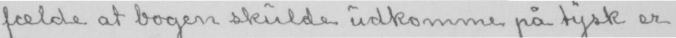fælde at bogen skulde udkomme på tysk er
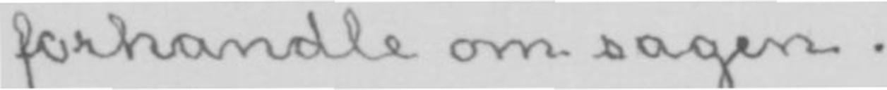forhandle om sagen.
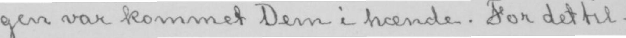gen var kommet Dem i hænde. For det til-
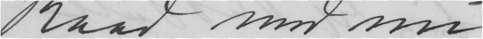Raad med nu.
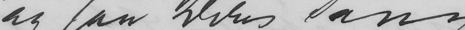og saa Deres Sang.
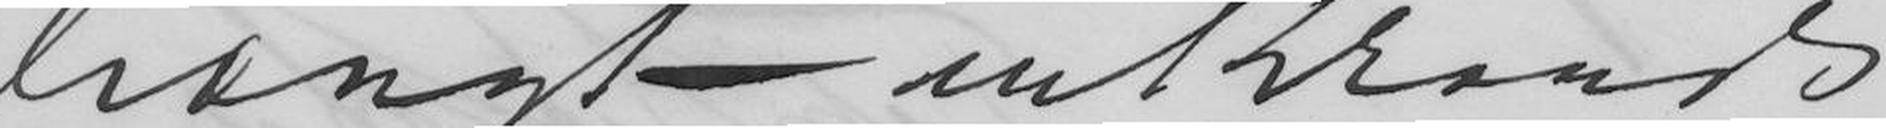hængt en Krands
